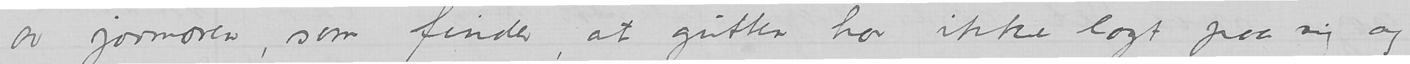av jormoren, som finder, at gutten har ikke lagt paa sig og
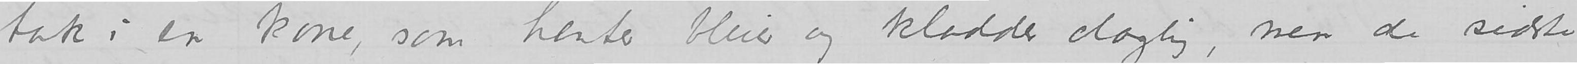tak i en kone, som henter bleier og kladder daglig, men de sidste
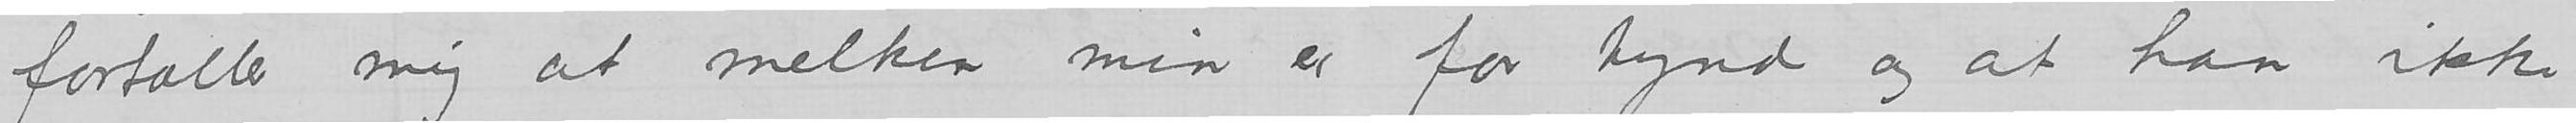fortæller mig at melken min er for tynd og at han ikke
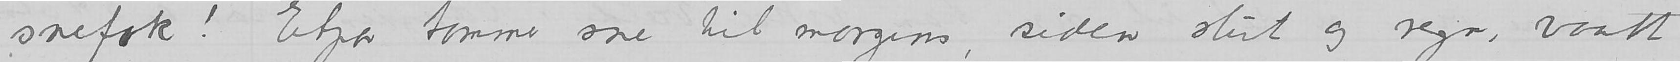snefok! Etpar tommer sne til morgens, siden slut og regn, vaatt
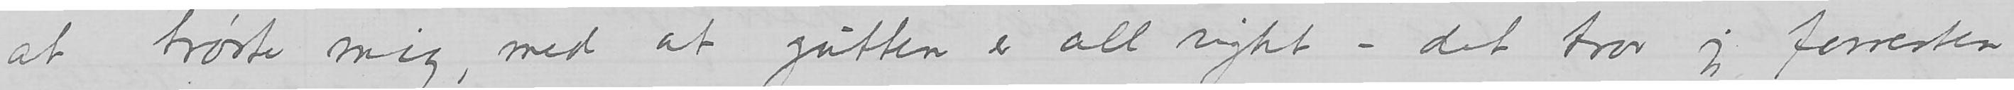at trøste mig, med at gutten er all right – det tror jeg forresten
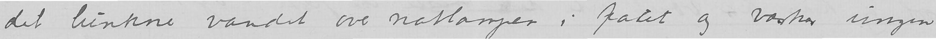det lunkne vandet over natlampen i taket og vasker ungen
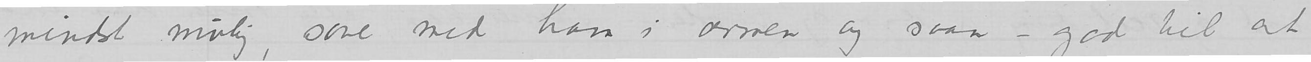mindst mulig, sove med ham i armen og saan – god til at
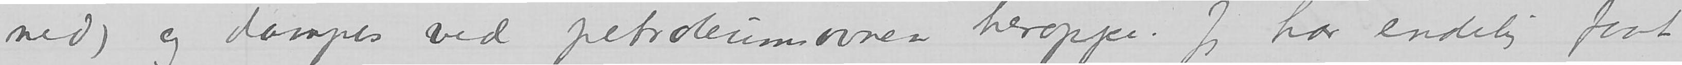ned) og dampes ved petroleumsovnen heroppe. Jeg har endelig faat
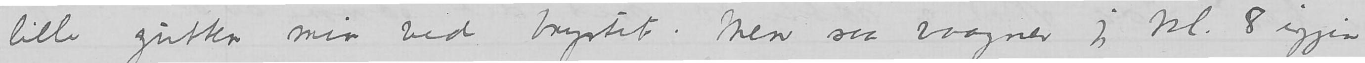lille gutten min ved brystet. Men saa vaagner jeg kl. 8 igjen
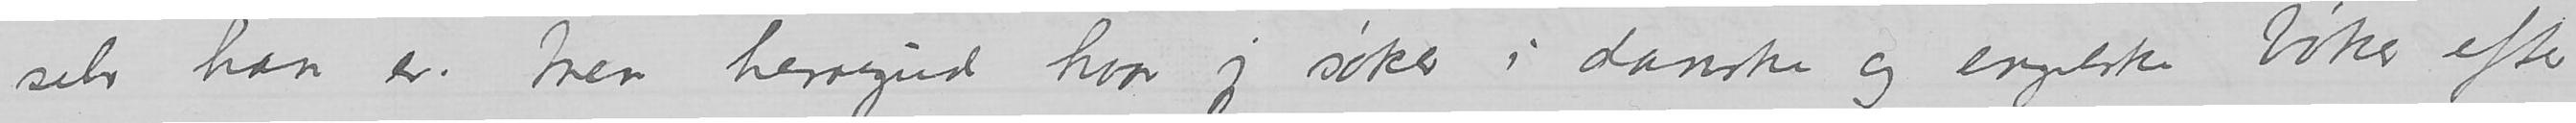selv han er. Men herregud hvor jeg søker i danske og engelske bøker efter
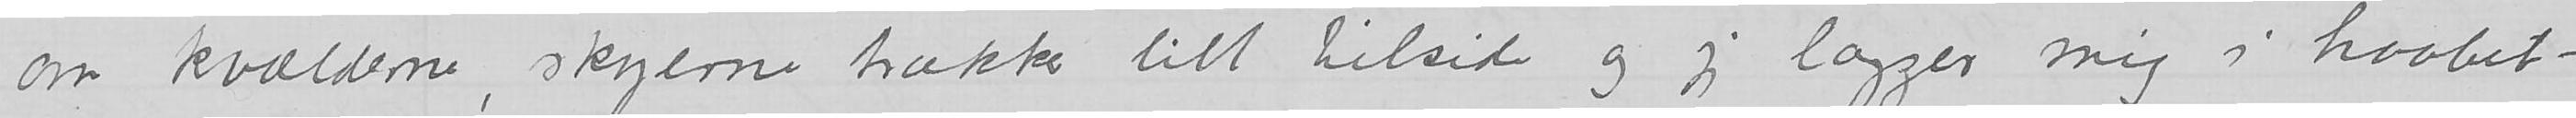om kvælderne, skyerne trækker litt tilside og jeg lægger mig i haabet –
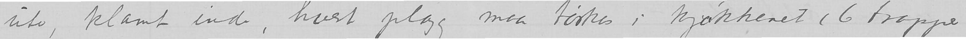ute, klamt inde, hvert plagg maa tørkes i kjøkkenet (6 trapper
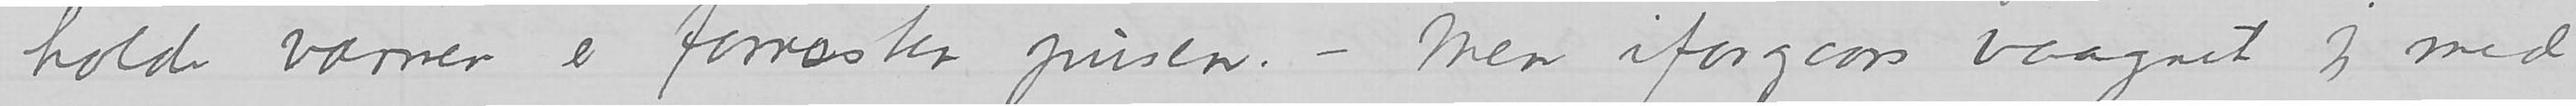holde varmen er forresten peisen. – Men iforgaars vaagnet jeg med
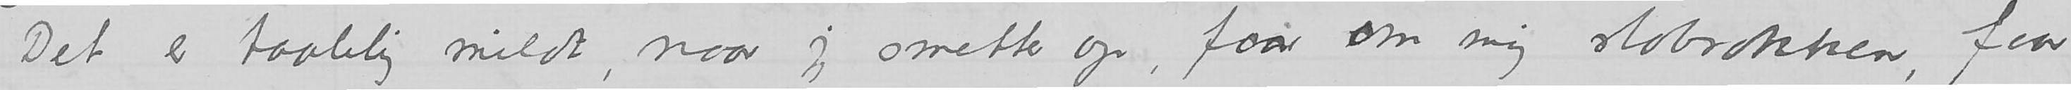Det er taalelig mildt, naar jeg smetter op, faar om mig slobrokken, faar
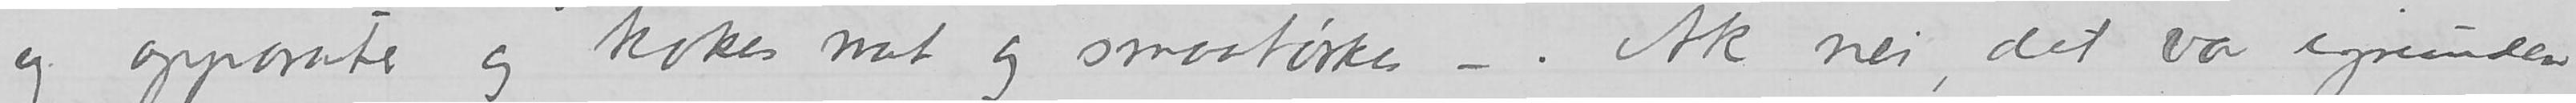og apparater og kokes mat og smaatørkes –. Ak nei, det var igrunden
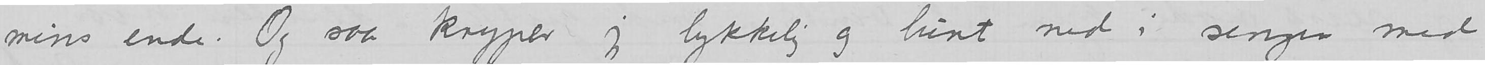mins ende. Og saa kryper jeg lykkelig og lunt ned i sengen med
